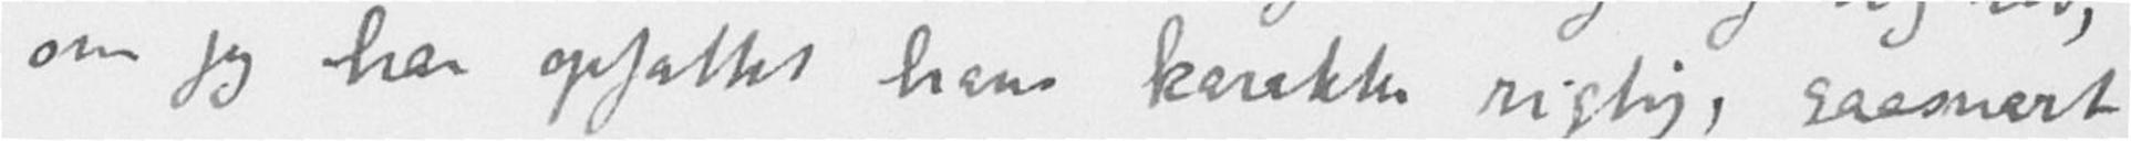om jeg har opfattet hans karakter rigtig, saasnart
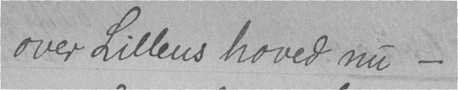over Lillens hoved nu -
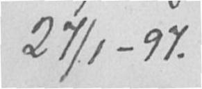27/1-97.
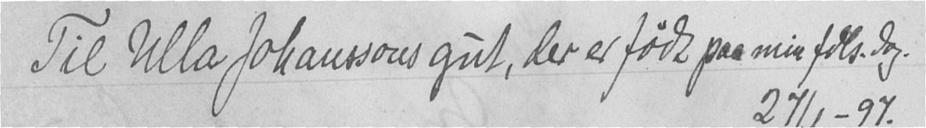Til Ulla Johanssons gut, der er født paa min føds. dag.
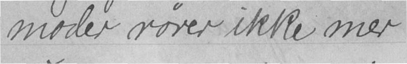moder rører ikke mer
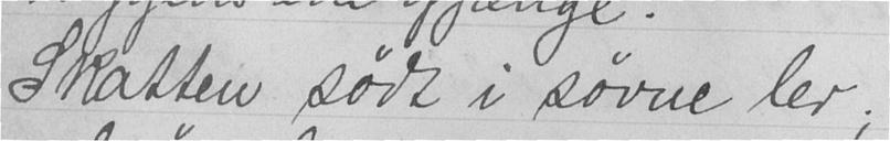Skatten sødt i søvne ler;
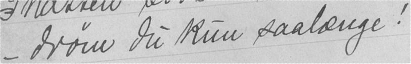- drøm du kun saalænge!
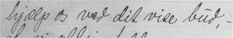hjælp os ved dit vise bud, -
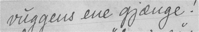vuggens ene gjænge!
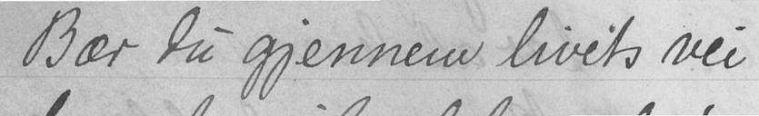Bær du gjennem livets vei
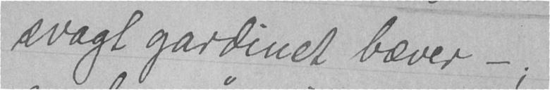svagt gardinet bæver - ;
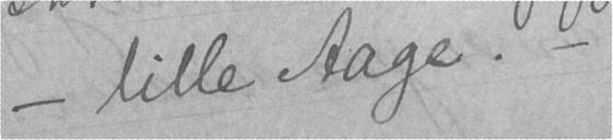- lille Aage. -
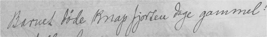Barnet døde knap fjorden dage gammel!
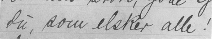du, som elsker alle!
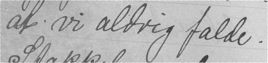at vi aldrig falde.
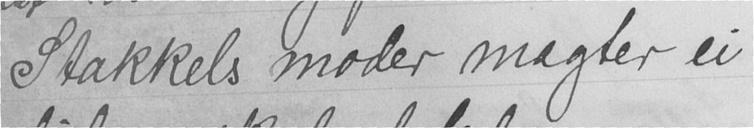Stakkels moder magter ei
